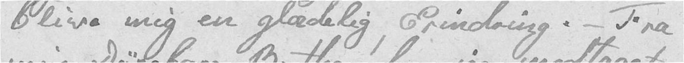blive mig en glædelig Erindring. – Fra
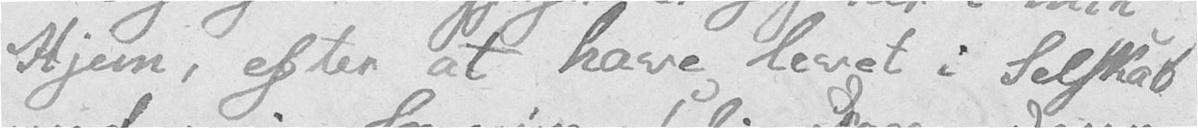Hjem, efter at have levet i Selskab
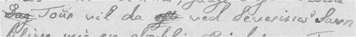Dag Tour vil da efte ved Severines Savn
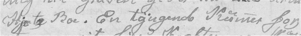Hjerte Roe. En tyngende Kummer for
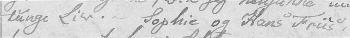tunge Liv. – Sophie og Hans Friis,
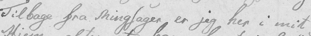Tilbage fra Ringsager er jeg her i mit
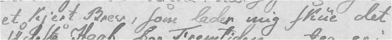et kjert Brev, som lader mig skue det
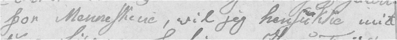for Menneskene, vil jeg hensukke mit
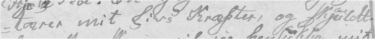-tærer mit Livs Kræfter, og skjuldt
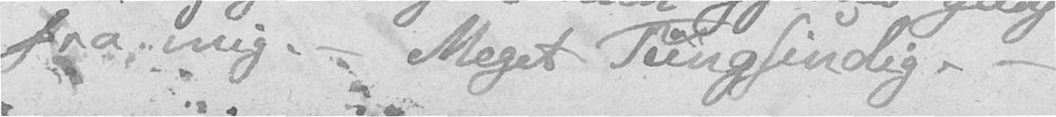fra mig. – Meget Tungsindig. –
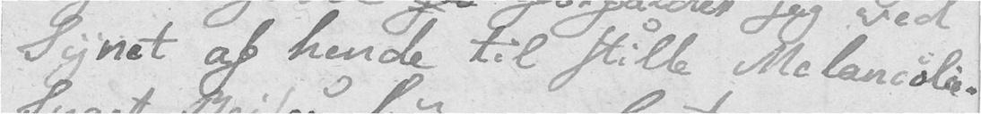Synet af hende til stille Melancolie.
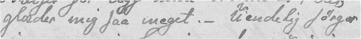glæder mig saa meget. – uendelig sørger
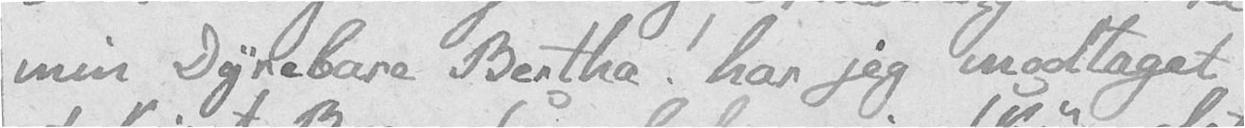min Dyrebare Bertha! har jeg modtaget
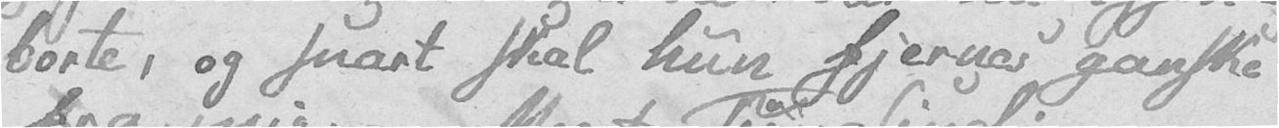borte, og snart skal hun fjernes ganske
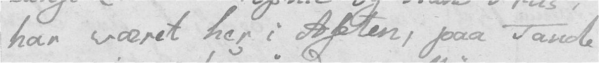har været her i Aften, paa Tande
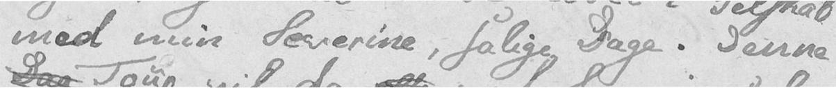med min Severine, salige Dage. Denne
